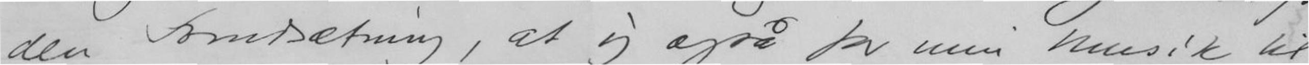- den Forudsætning, at jeg også for min Musik til
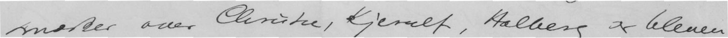mærker over Christie, Kjerulf, Holdberg er bleven
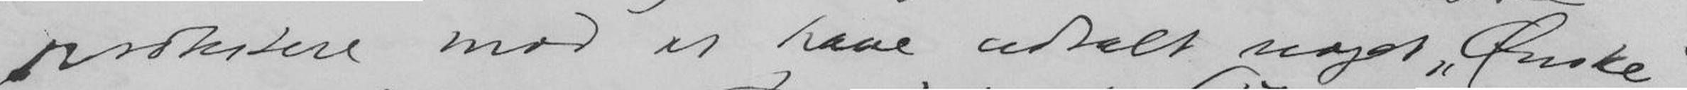protestere mod at have udtalt noget Ønske
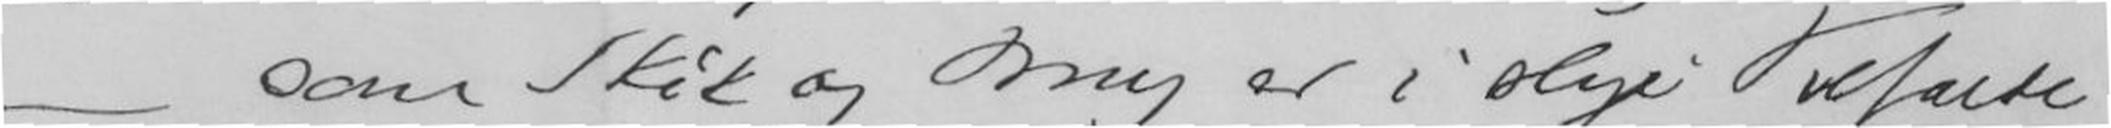honoreret - som Skik og Brug er i slige Tilfælde -
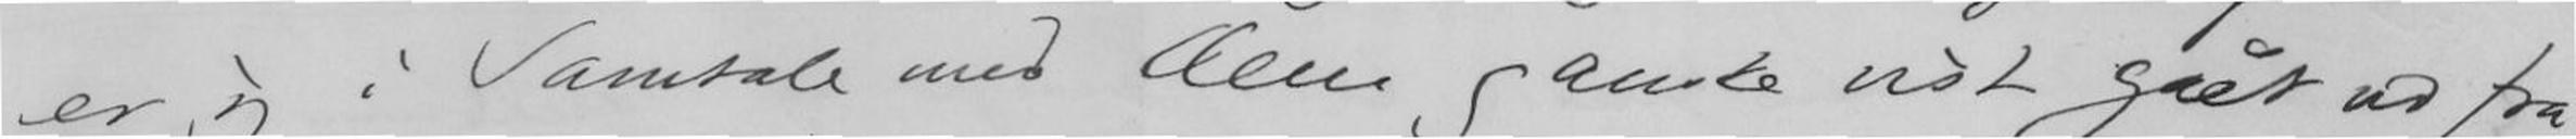er jeg i Samtale med Dem ganske vist gået ud fra
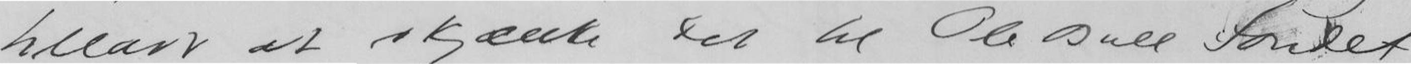tilladt at skjænke det til Ole Bull Fondet.
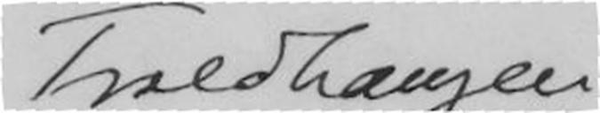Troldhaugen
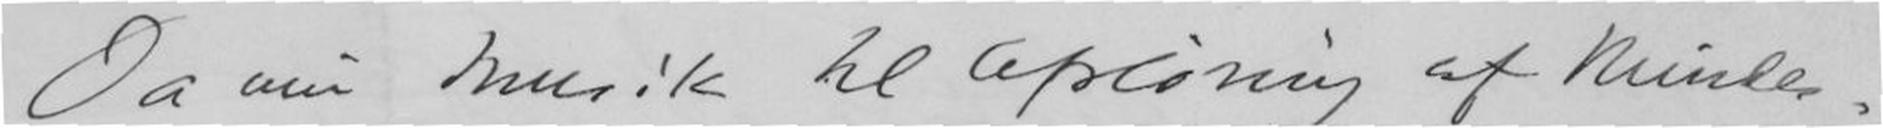Da min Musik til Afsløring af Mindes-
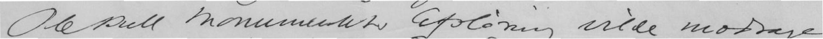Ole Bull Monumentets Afsløring vilde modtage
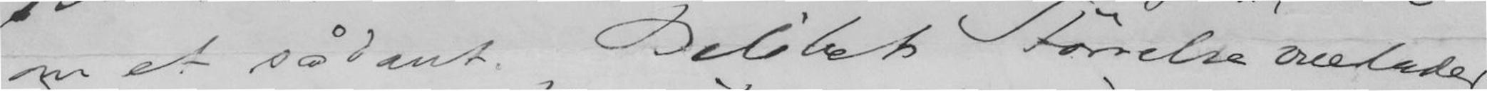om et sådant. Beløbets Størrelse overlader
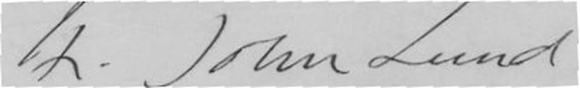Hr John Lund
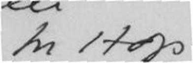pr Hop
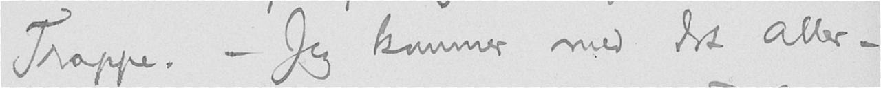Trappe. - Jeg kommer med det aller-
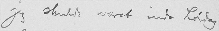jeg skulde været inde Lørdag
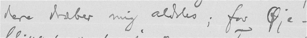dere dræber mig aldeles; for Øje-
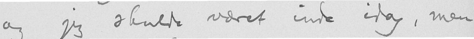og jeg skulde været inde idag, men
